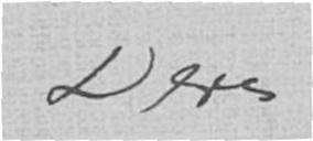Deres
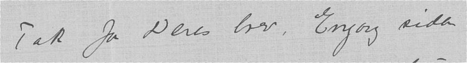Tak for Deres brev. Engang siden
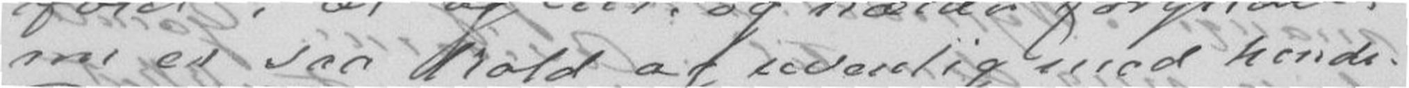nu er saa koldt og uvenlig mod hende.
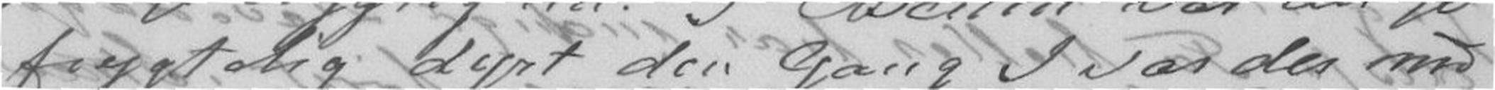frygtelig dyrt den Gang I var der med
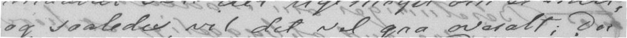og saaledes vil det gaa overalt; Det
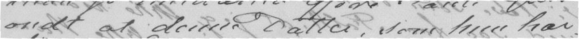ondt at denne Datter, som hun har
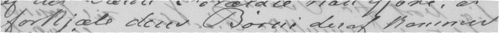forkjæle dens Børne deraf kommer
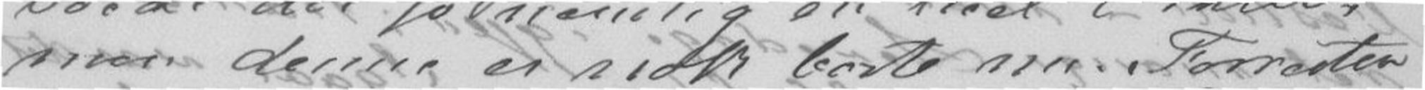men denne er nok borte nu. Forresten
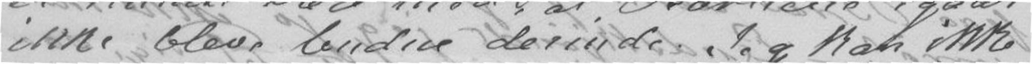ikke bleve budne derinde. Jeg kan ikke
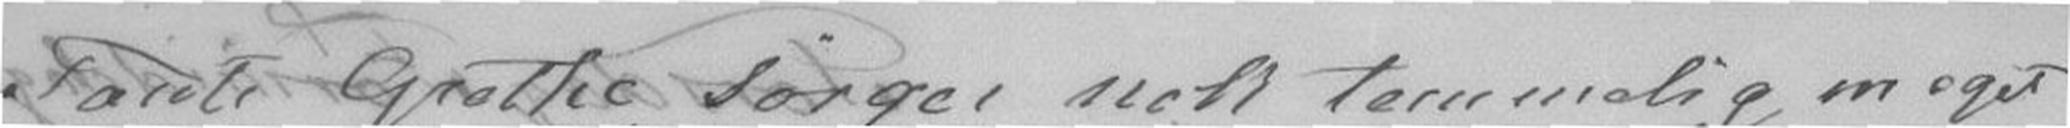Tante Grethe sørger nok temmelig meget
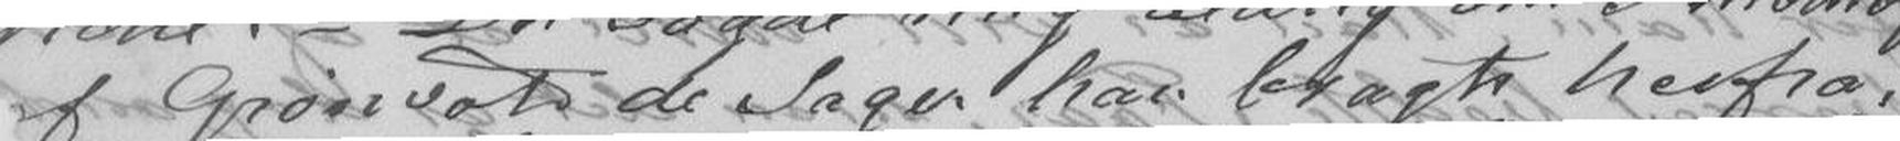af Grønvold de Sager han bragte herfra
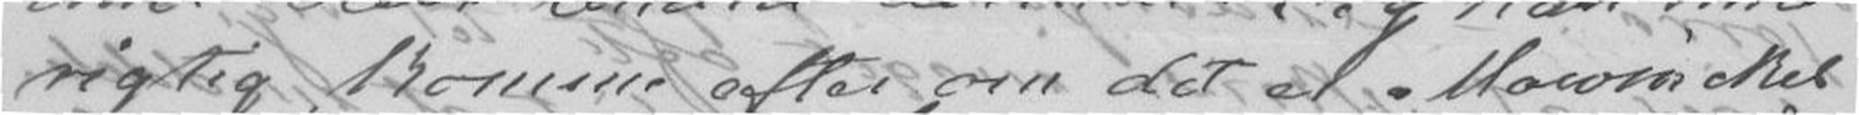rigtig komme efter om det er Mowinckel
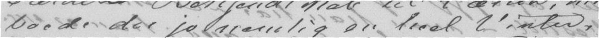boede der jo nemlig en heel Vinter,
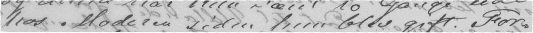hos Moderen siden hun blev gift. For-
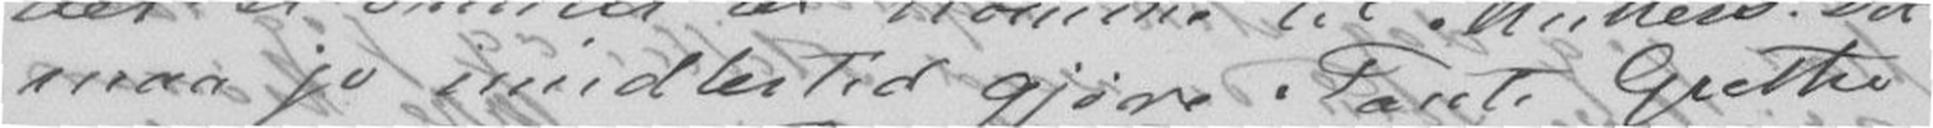maa jo imidlertid gjøre Tante Grethe
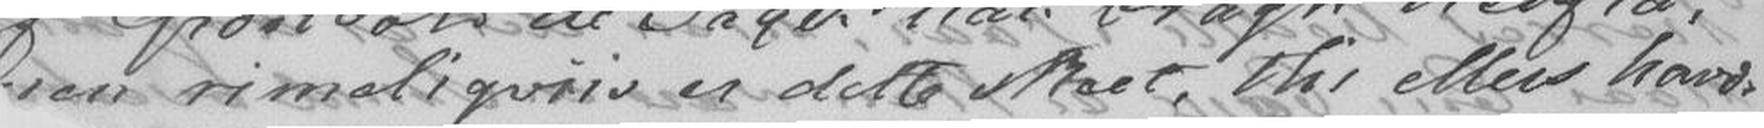en rimeligviis er dette skeet, thi ellers havde
